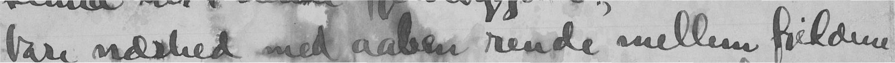bare nærhed med aaben rende mellem fjeldene
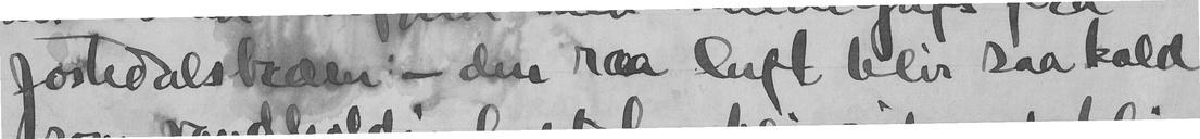Jostedalsbræen; - den raa luft blir saa kald
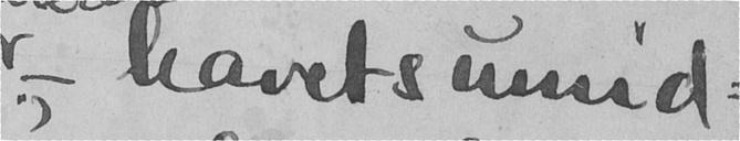, - havets umid-
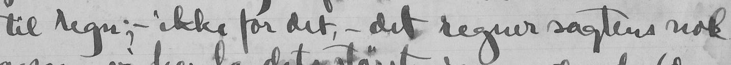til regn; - ikke for det, - det regner sagtens nok
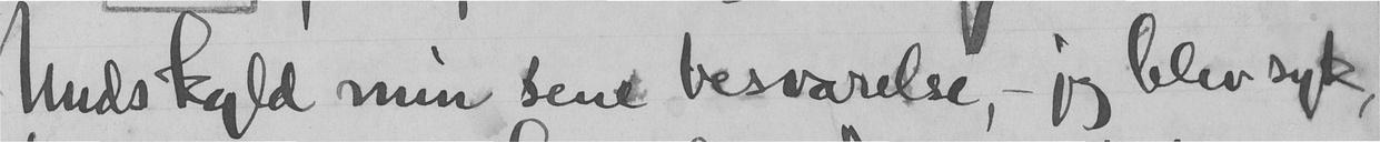Undskyld min sene besvarelse - jeg blev syk,
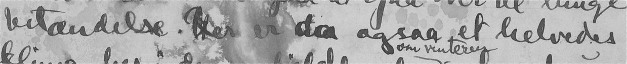betændelse. Her er da ogsaa et helvedes
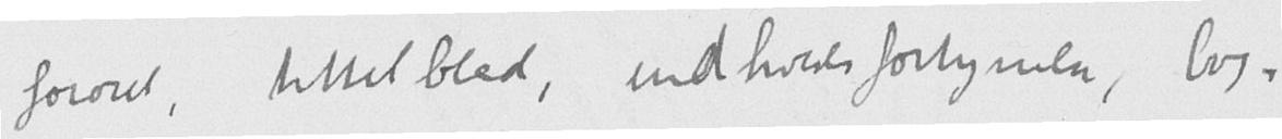forord, tittelblad, indholdsfortegnelse, bog-
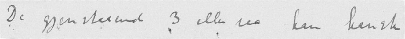De gjenstaaende 3 eller saa kan kanske
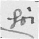før
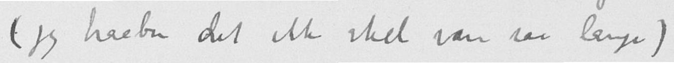(jeg haaber det ikke skal være saa længe)
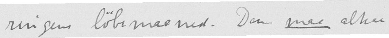ringens løbemaaned. Den maa altsaa
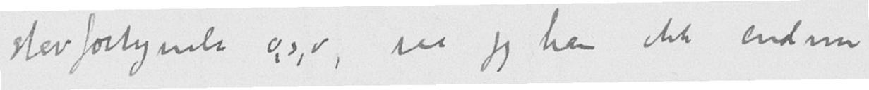stavfortegnelse o.s.v. saa jeg har ikke endnu
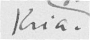Kria.
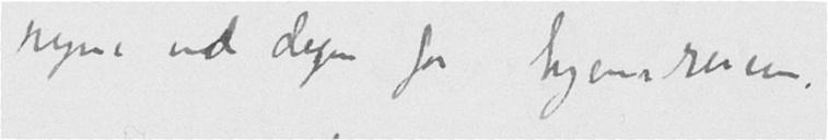regnet ud dagen for hjemreisen.
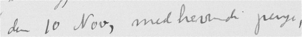den 10 Nov, med hevredi penge,
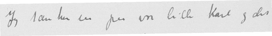Jeg tænker saa paa vor lille Karl og det
